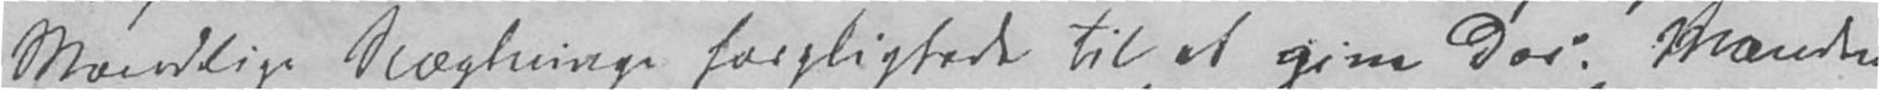Mandlige Slægtninger forpligtede til at give dar. Manden
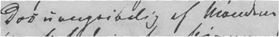Dos uangribelig af Mandens
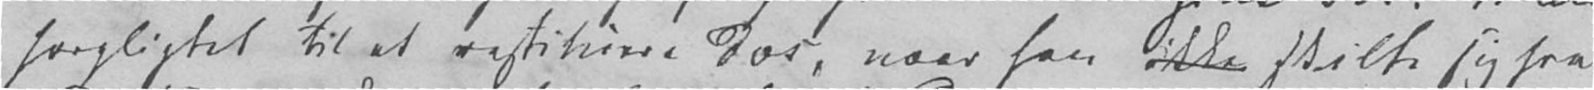forpligtet til at restituere Dor, naar han ikke skilte sig fra
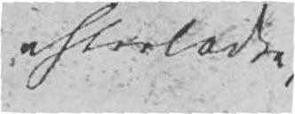efterladte,
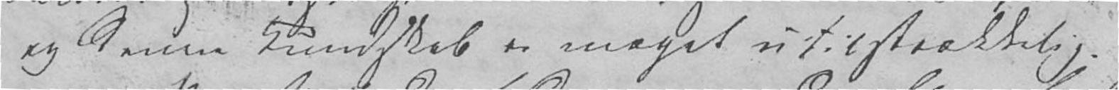og denne Kundskab er meget utilstrækkelig.
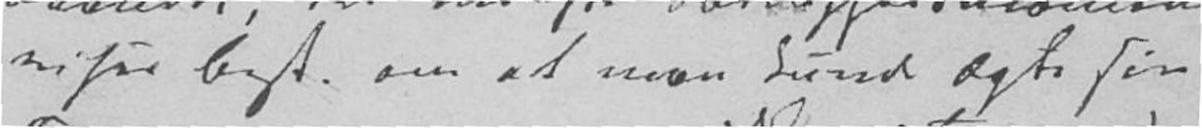viser Best. om at man kunde ægte sin
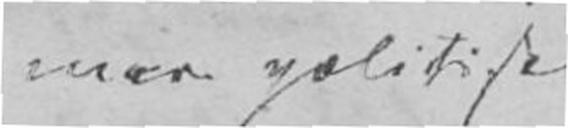mere politisk
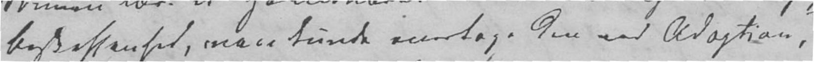Beskaffenhed, man kunde overtage den ved Adoption,
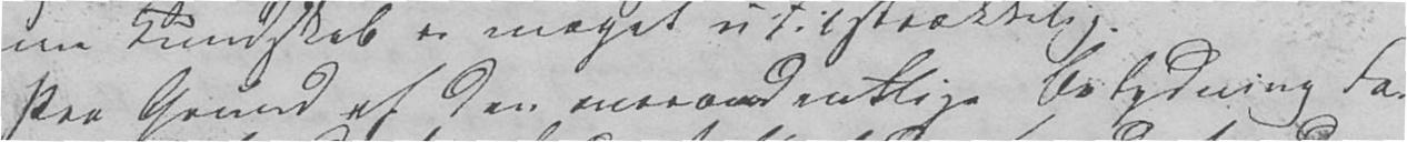Paa Grund af den overordentlige Betydning Fa-
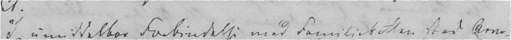I umiddelbar Forbindelse med Familieretten stod Arve-
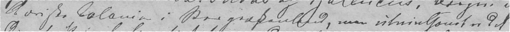doriske Colonier i Storgrækenland, men utvivlsomt er det
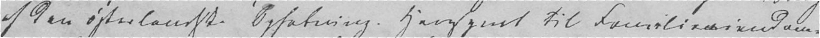af den østerlandske Opfatning. Hensynet til Familieiendom-
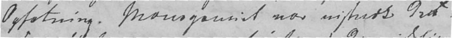Opfatning. Monogamiet var vistnok den
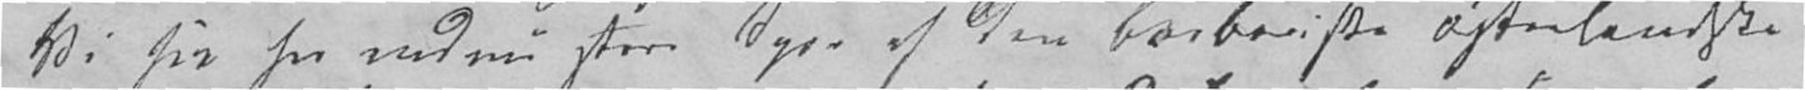Vi ser her endnu store Spor af den barbariske østerlandske
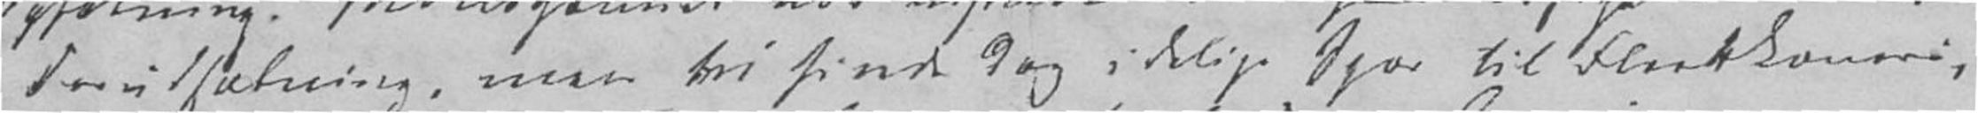Forudsætning, men vi finde dog idelige Spor til Fleerkoneri,
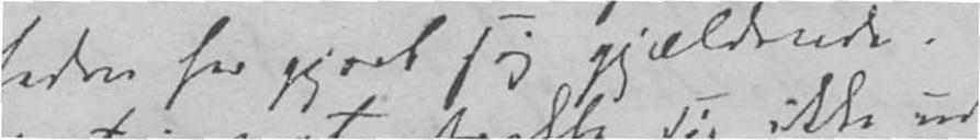heden har gjort sig gjældende.
